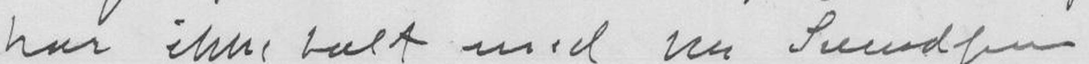har ikke talt med hr. Svendsen
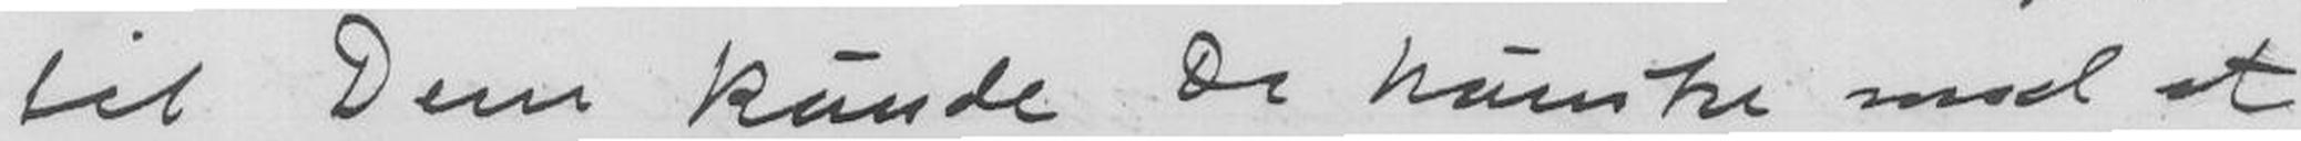til Dem kunde De kanske med et
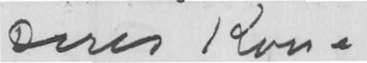Deres Kone
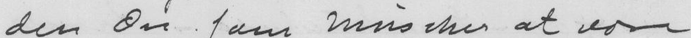den Ære som musiker at være
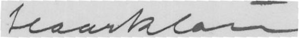J. Haarklau
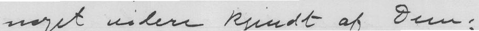noget videre kjendt af Dem;
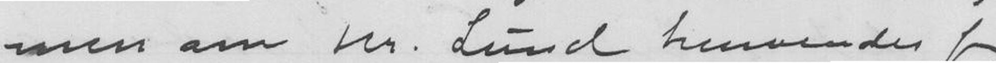men om hr. Lund henvender sig
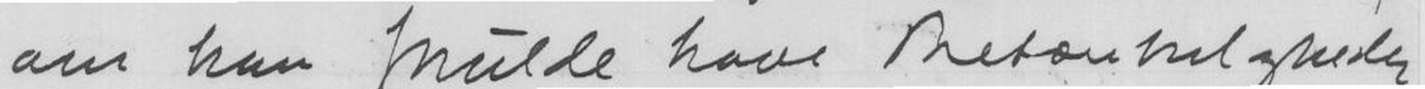om han skulde have Betænkeligheder
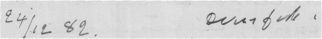24/12 82. Deres forb.
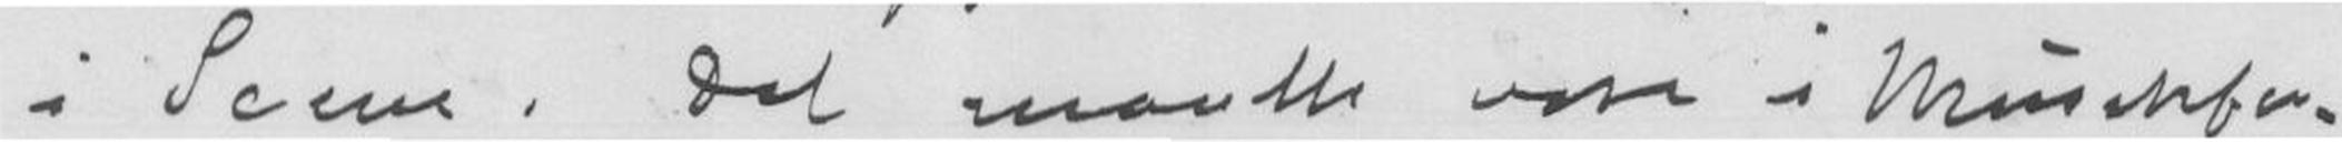i Scene. Det maatte være i Musikfor-
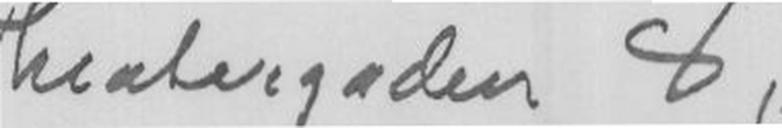Theatergaden 8
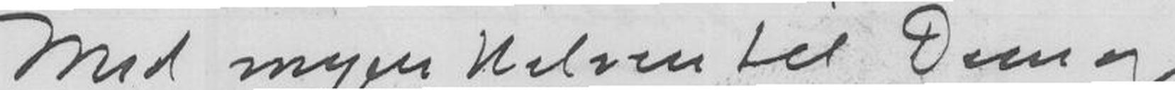Med megen Hilsen til Dem og
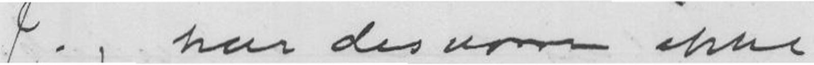Jeg har desværre ikke
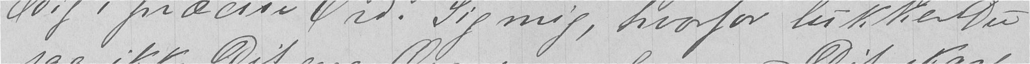Dig i præcise Ord! Sig mig, hvorfor lukker Du
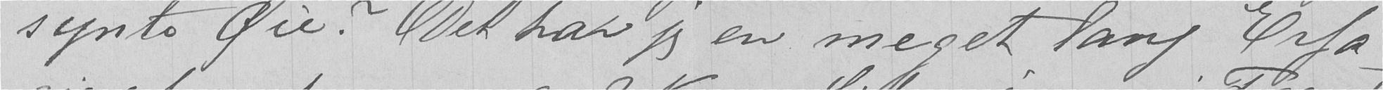synte Øie? Det har jeg en meget lang Erfa-
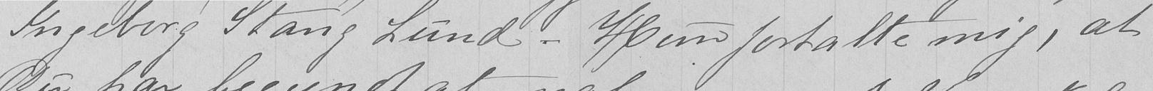Ingeborg Stang Lund. Hun fortalte mig, at
